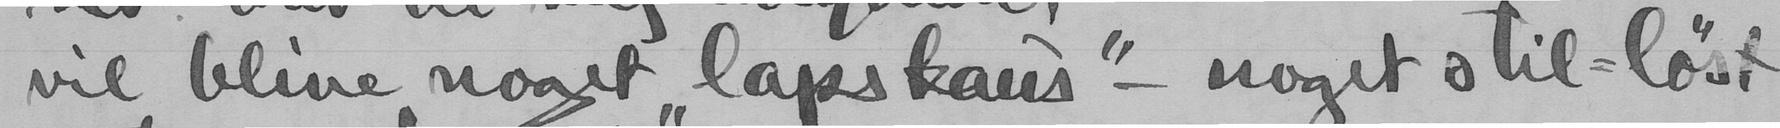vil blive noget "lapskaus" - noget stil-løst
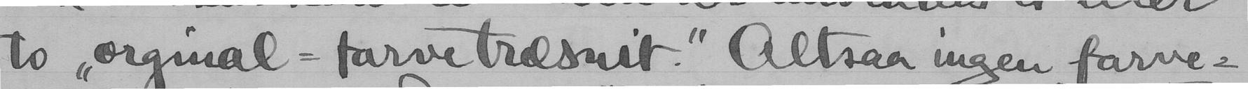to "orginal-farvetræsnit". Altsaa ingen farve-
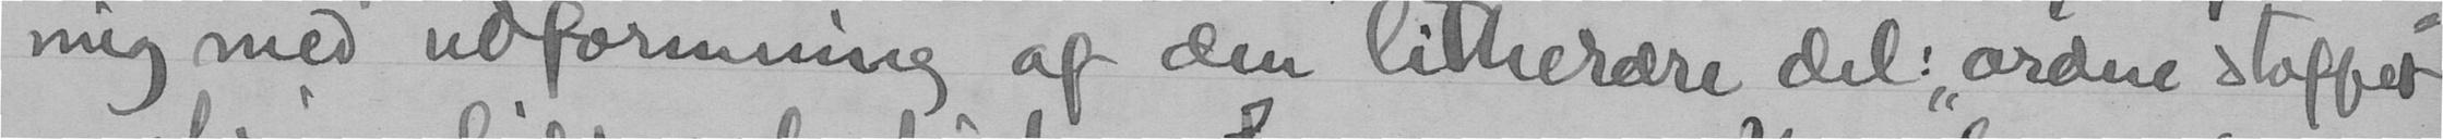mig med udformnning af den litherære del: "ordne stoffet"
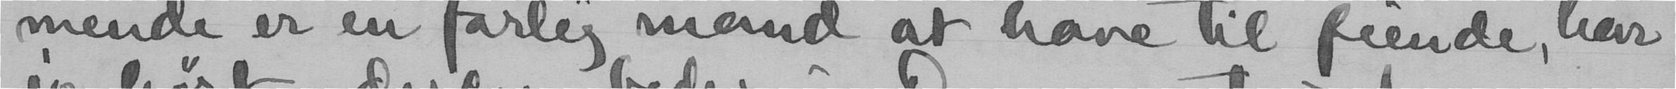mende er en farlig mand at have til fiende, har
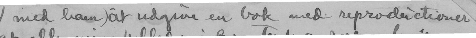med ham at udgive en bok med reproductioner
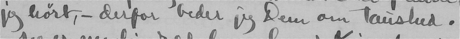jeg hørt, - derfor beder jeg Dem om taushed.
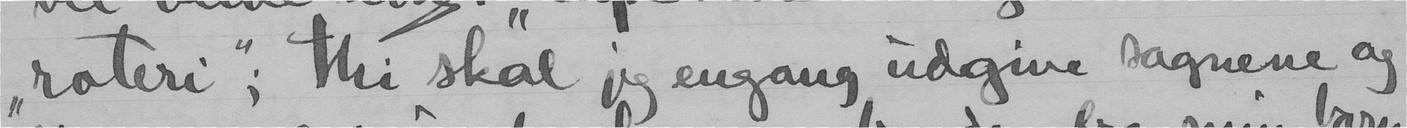"roteri"; thi skal jeg engang udgive sagnene og
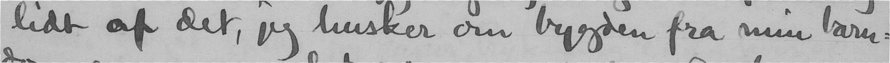lidt af det, jeg husker om bygden fra min barn-
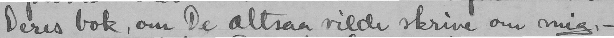Deres bok, om De altsaa vilde skrive om mig, -
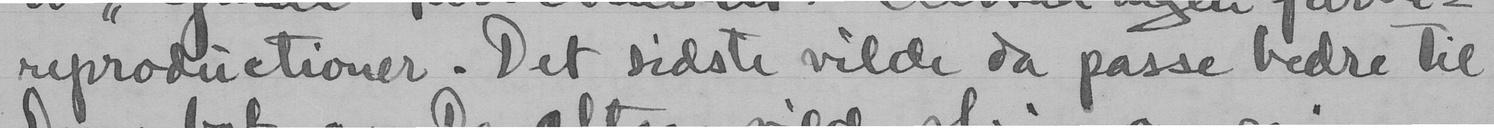reproductioner. Det sidste vilde da passe bedre til
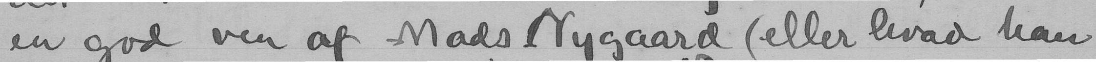en god ven af Mads Nygaard (eller hvad han
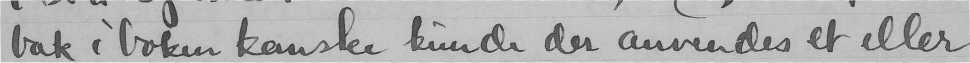bak i boken kanske kunde der anvendes et eller
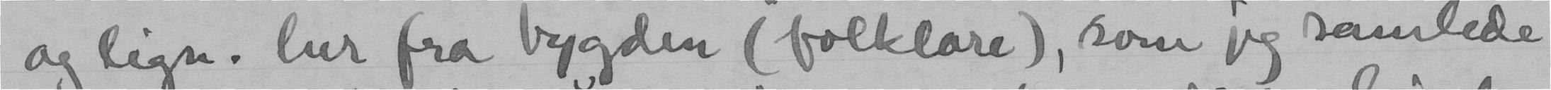og lign. her fra bygden (folklore), som jeg samlede
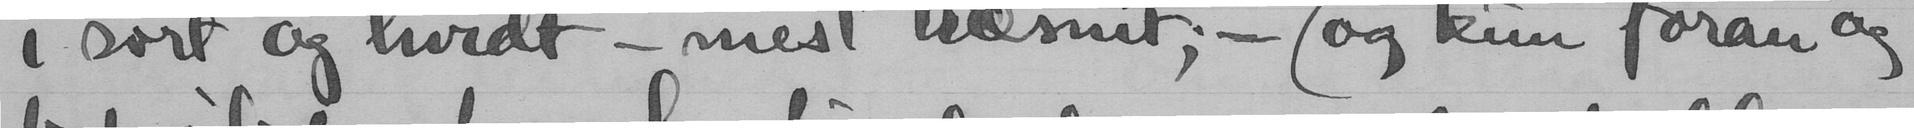i sort og hvidt - mest træsnit, - (og kun foran og
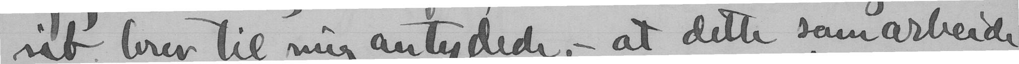sit brev til mig antydede, - at dette samarbeide
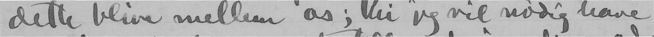dette blive mellem os; thi jeg vil nødig have
